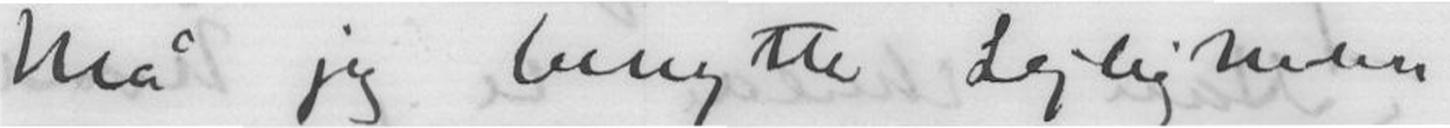Må jeg benytte Lejligheden
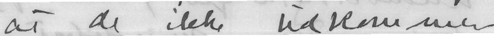at de ikke udkommer
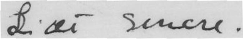Lidt senere.
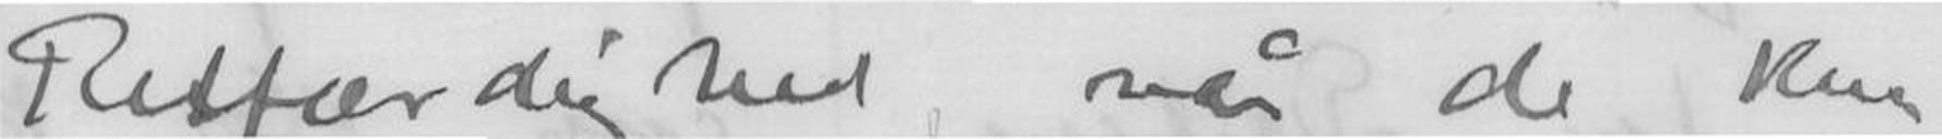Retfærdighed, når de kun
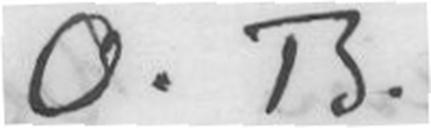O.B.
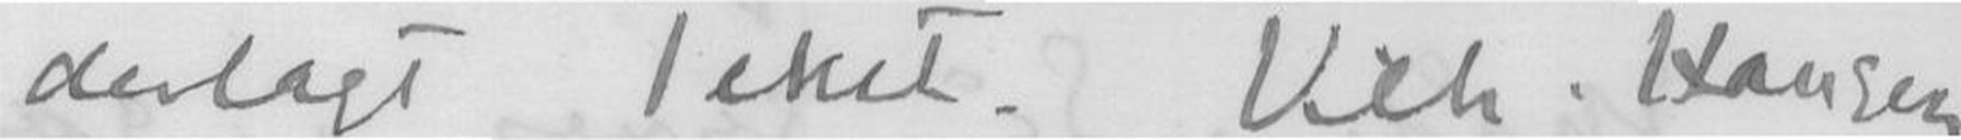derlagt Tekst. Vilh. Hansen
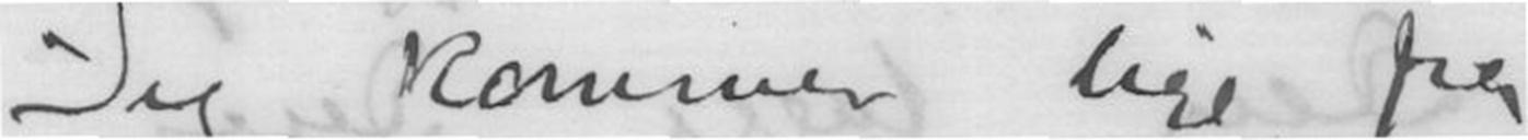Jeg kommer lige fra
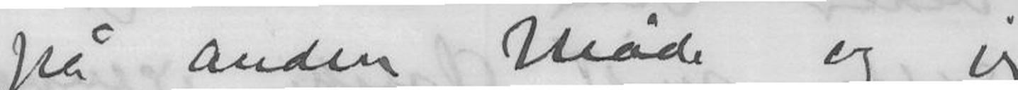på anden Måde og jeg
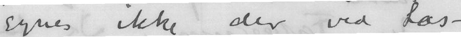synes ikke der ved Læs-
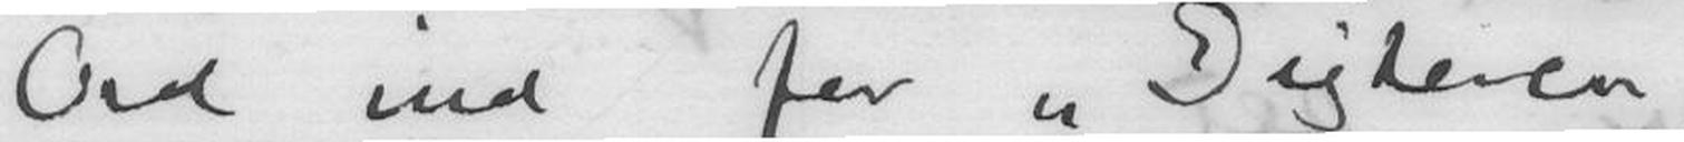Ord ind for Digderen
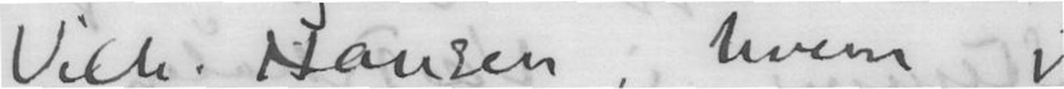Vilh. Hansen, hvem jeg
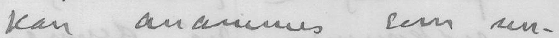kan anammes som un-
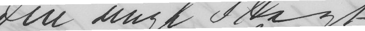den unge Slægt
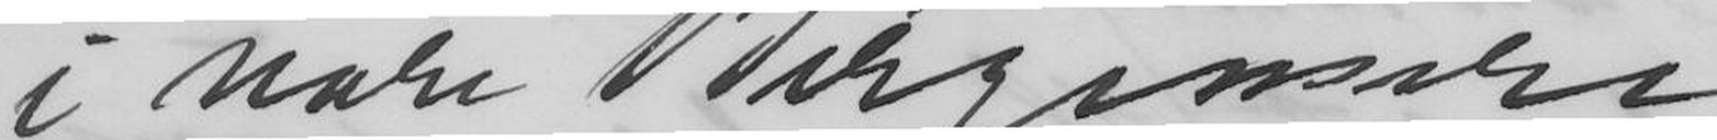i vore Bergensere
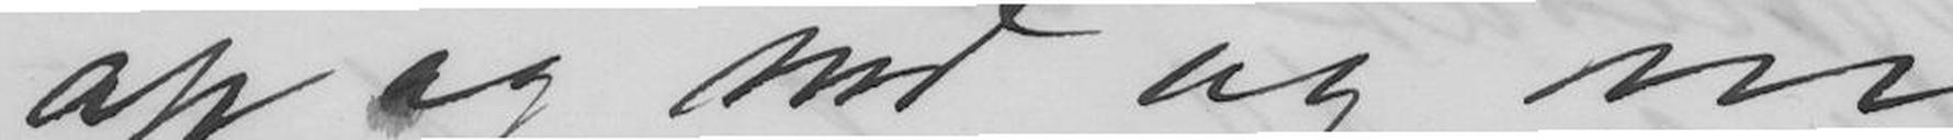op og ned og vi
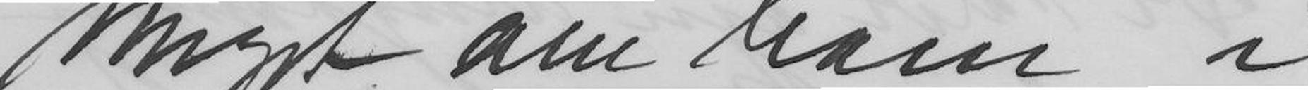meget om ham i
